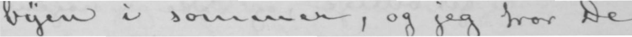byen i sommer, og jeg tror De
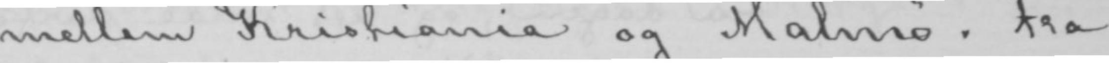mellem Kristiania og Malmø. Fra
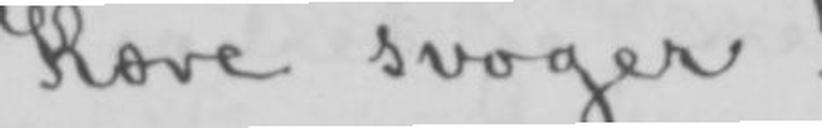Kære svoger!
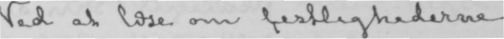Ved at læse om festlighederne
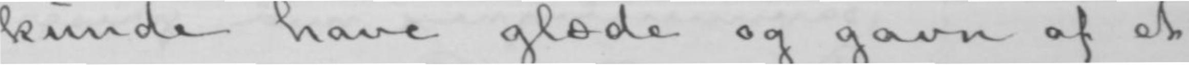kunde have glæde og gavn af et
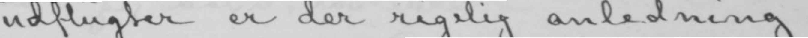udflugter er der rigelig anledning.-
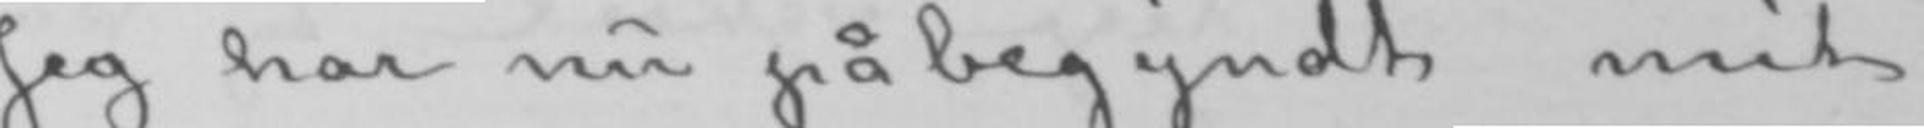Jeg har nu påbegyndt mit
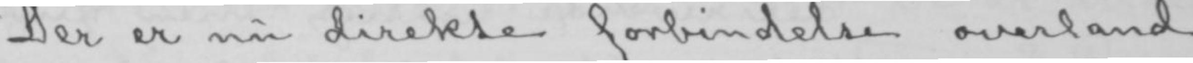Der er nu direkte forbindelse overland
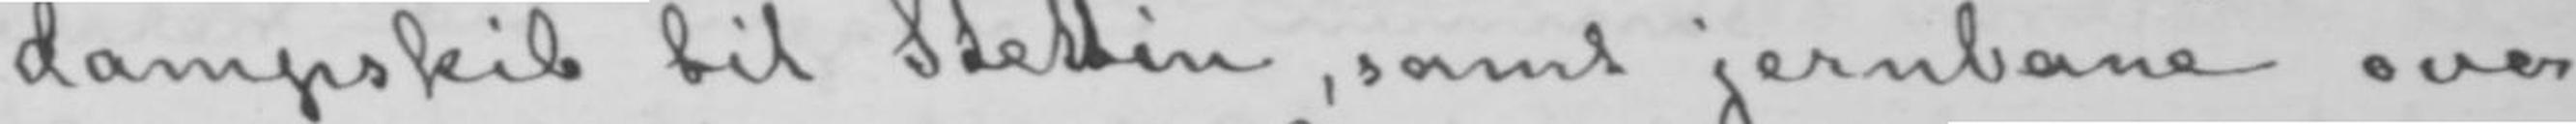dampskib til Stettin, samt jernbane over
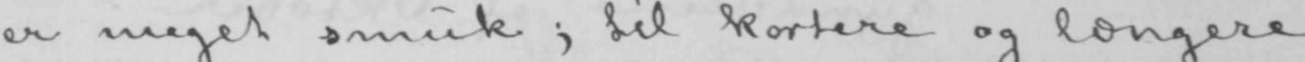er meget smuk; til kortere og længere
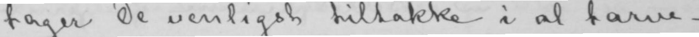tager De venligst tiltakke i al tarve-
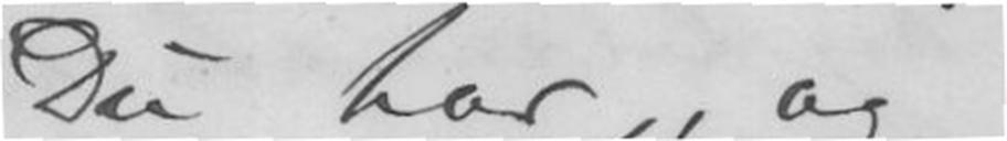Du har, og
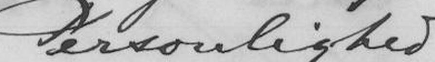Personlighed
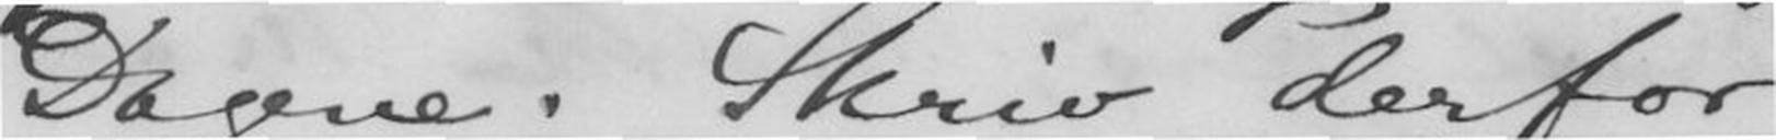Dagene. Skriv derfor
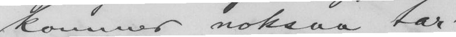kommer noksaa tar
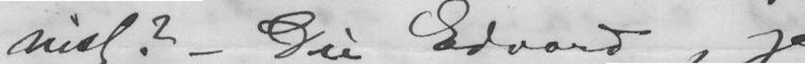nist? - Du Edvard, jeg
